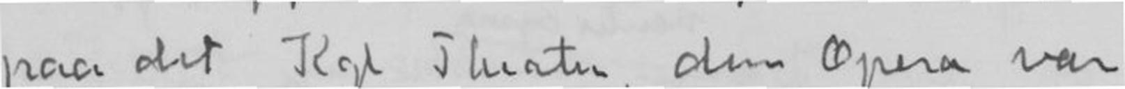paa det Kgl Theater, den Opera var
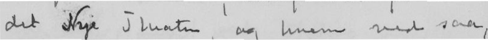det Nye Theater, og hvem ved saa,
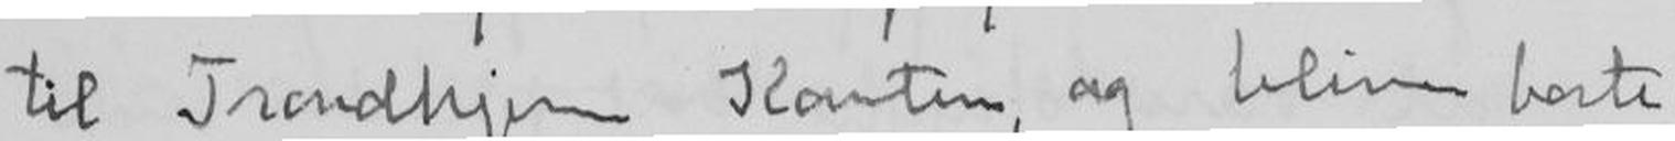til Trondhjem Kanten, og bliver borte
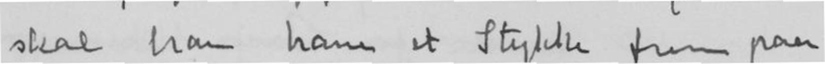skal han have et Stykke frem paa
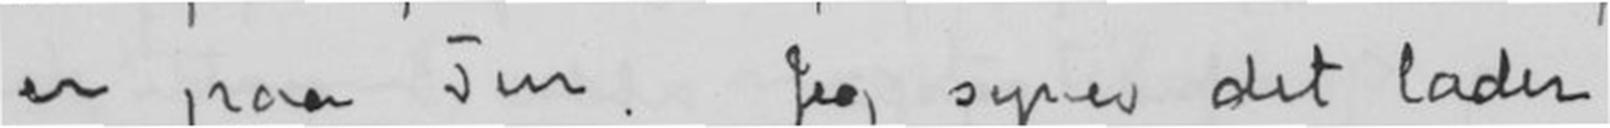er paa Tur. Jeg syntes det lader
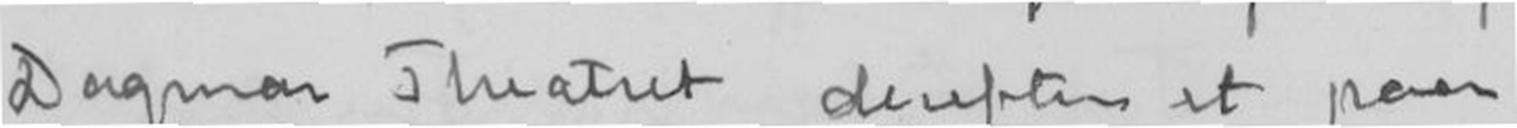Dagmar Theateret, derefter et paa
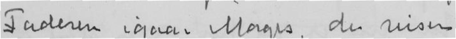Faderen igaar Morges, de reiser
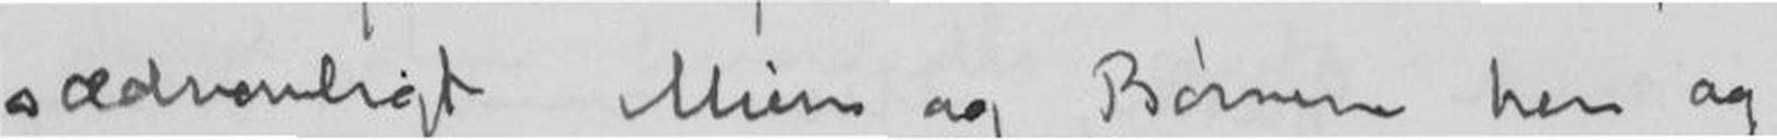sædvenligt Mien og Børnene her og
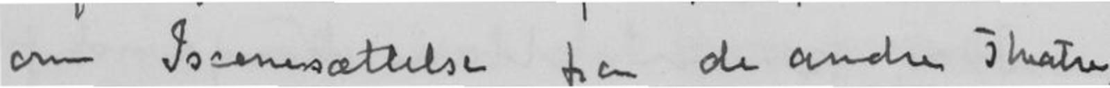om Iscenesættelse fra de andre Theatre,
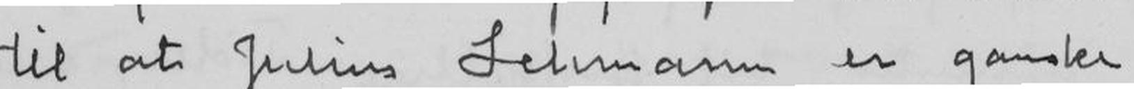til at Julius Selmann er ganske
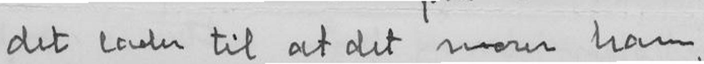det lader til at det urorer ham
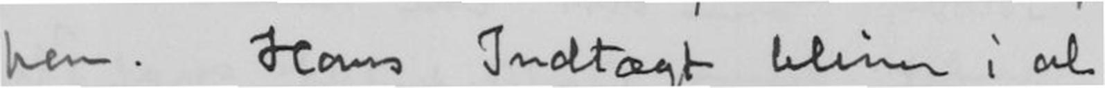hen. Hans Indtægt bliver i al
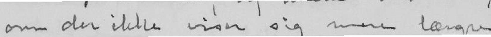om der ikke viser sig mere længre
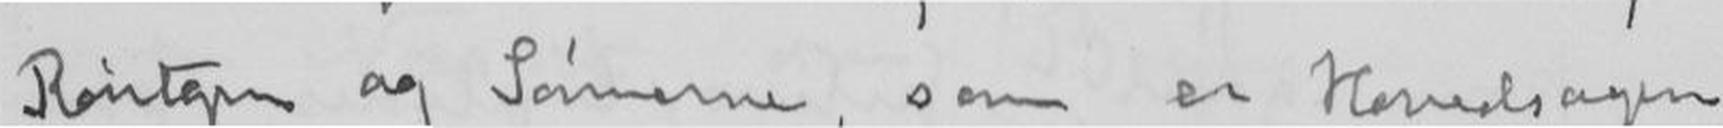Röntger og Sønnerne, som er Hovedsagen
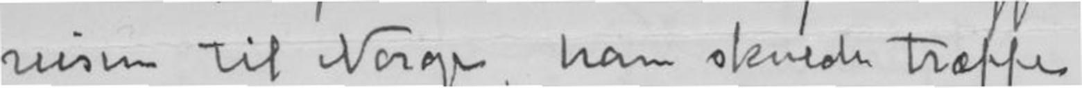reise til Norge, han skulde træffe
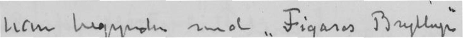han begynte ved "Figaros Brullup"
